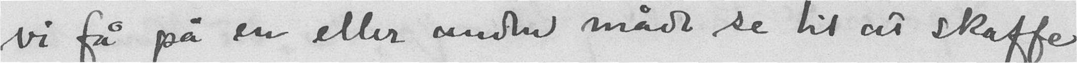vi får på en elller anden måde se til at skaffe
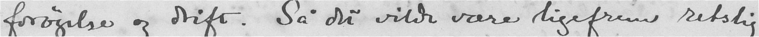forøgelse og drift. så det vilde være ligefrem retslig
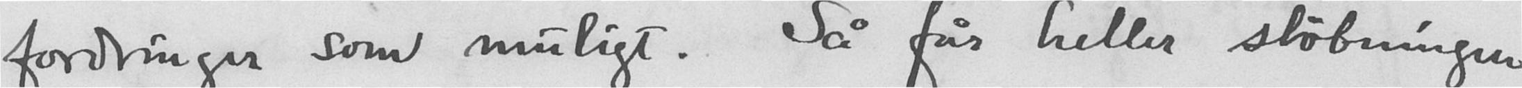fordringer som muligt. Så får heller stæbningen
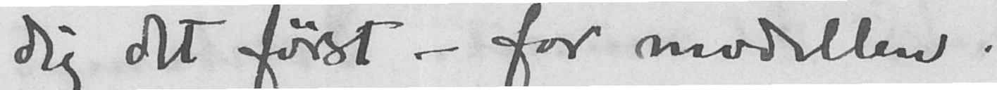dig det først - for modellen.
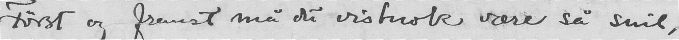Først og fremst må du vistnok være så snil,
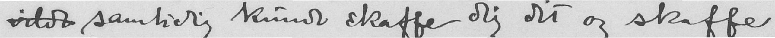vilde samtidig kunde skaffe dig dit og skaffe
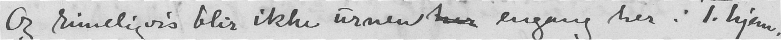Og rimeligvis blir ikke urnen her engang her i T. hjem.
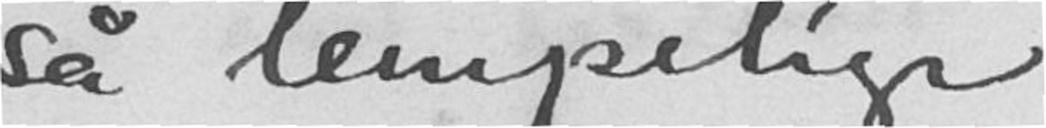så lempelige
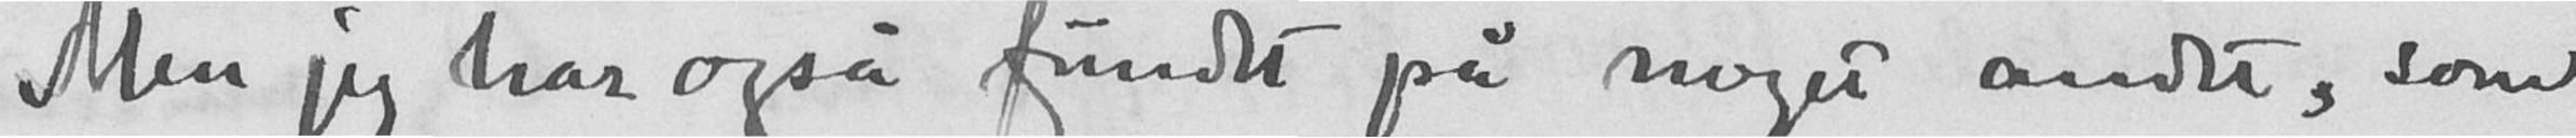Men jeg har også fundet på noget andet, som
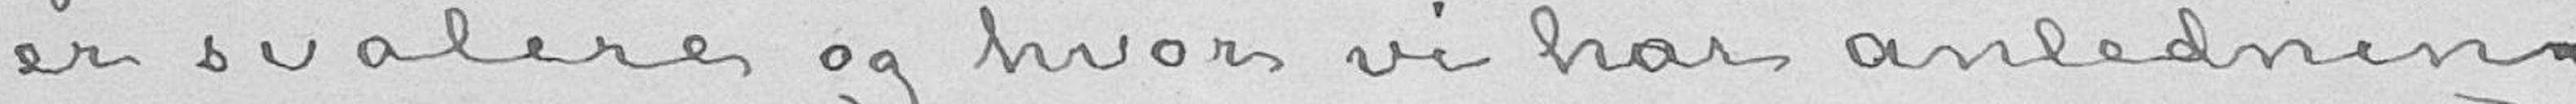er svalere og hvor vi har anledning
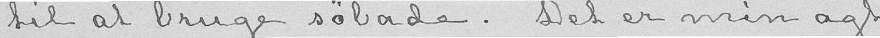til at bruge søbade. Det er min agt
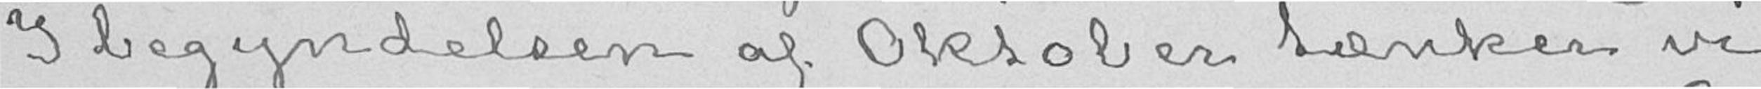I begyndelsen af Oktober tænker vi
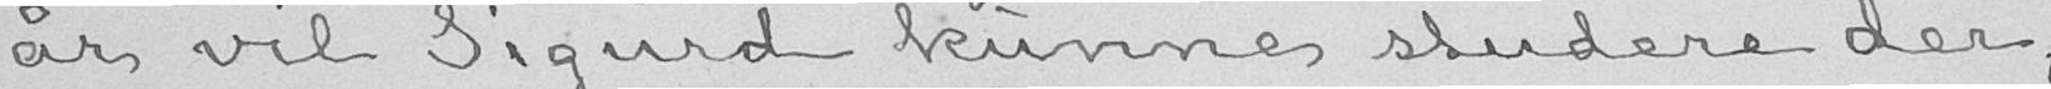år vil Sigurd kunne studere der,
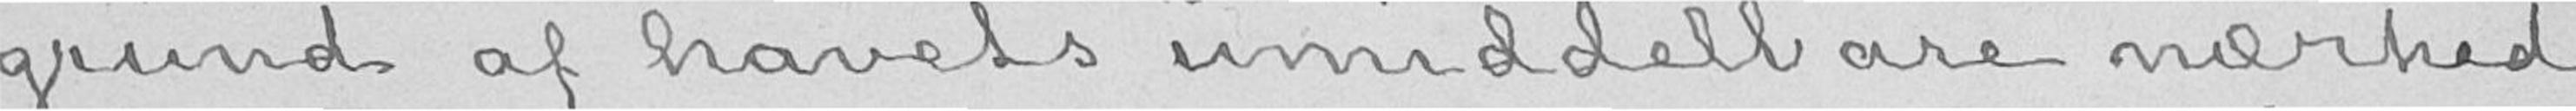grund af havets umiddelbare nærhed
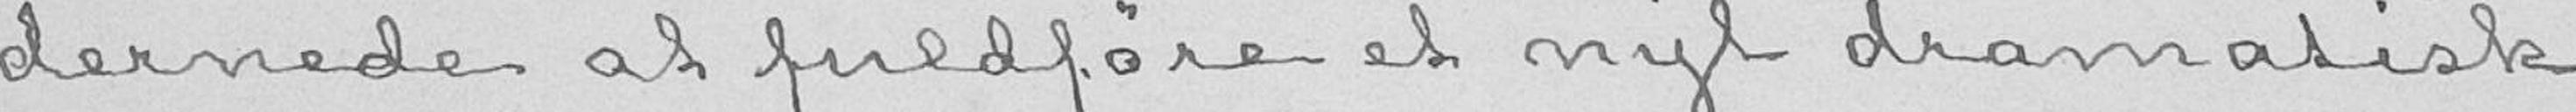dernede at fuldføre et nyt dramatisk
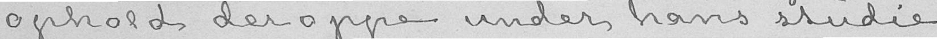ophold deroppe under hans studie-
